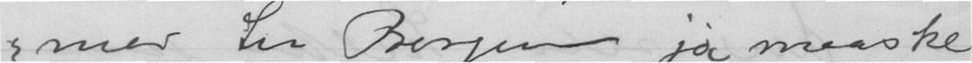mer til Bergen ja maaske
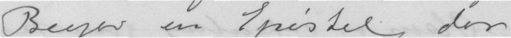Beyer en Epistel der
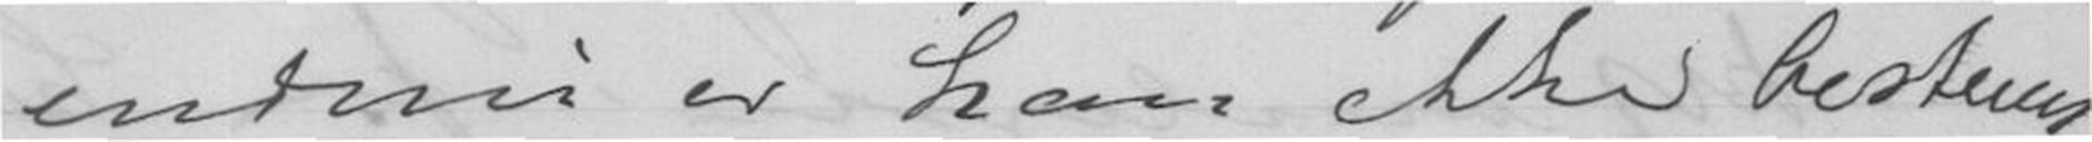endnu er han ikke bestemt
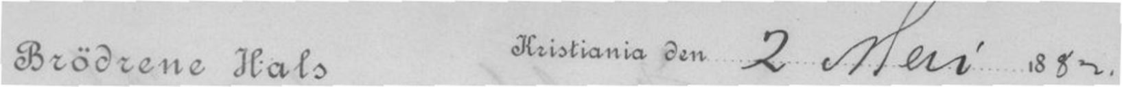Brødrene Hals Kristiania den 2 Mai 1882
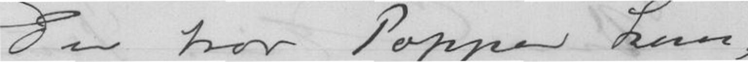Du tror Poppe kom
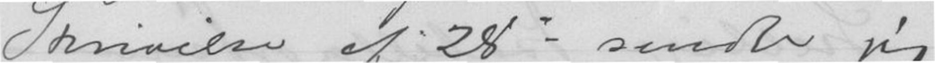Skrivelse af 28de sendte jeg
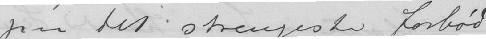paa det strengeste forbød
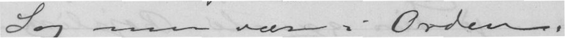Sag maa være i Orden.
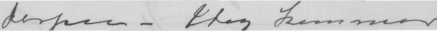derpaa. Idag kommer
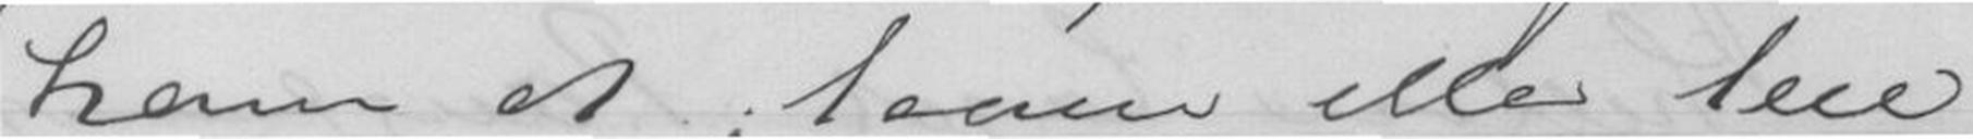ham at laane eller leie
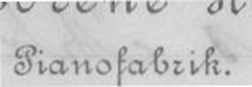Pianofabrik.
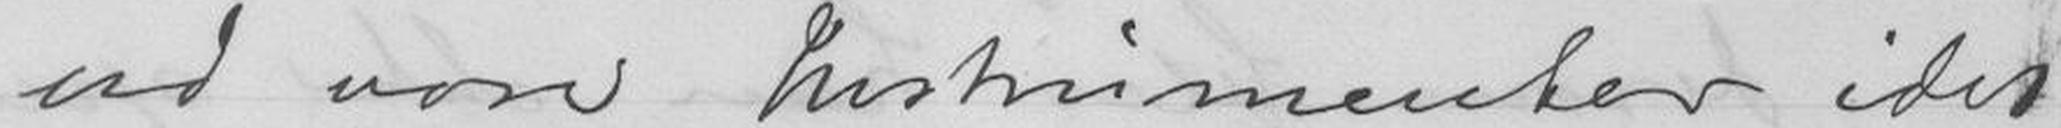ud vore Instrumenter idet
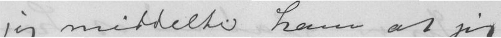jeg meddelte ham at jeg
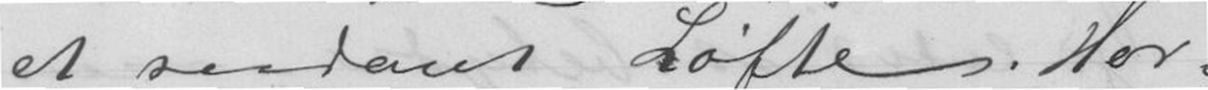et saadant Løfte. Her-
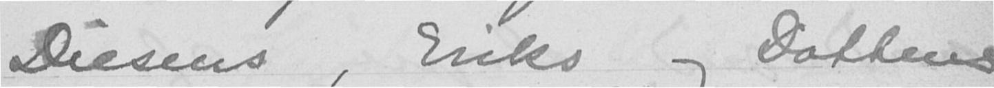Diesens, Eriks og Dottens
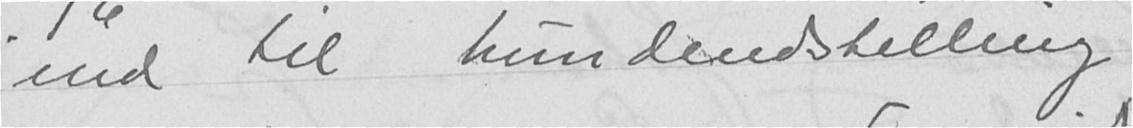ind til hundeudstilling
